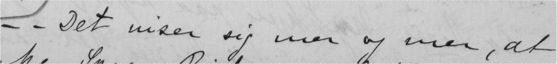- - Det viser sig mer og mer, at
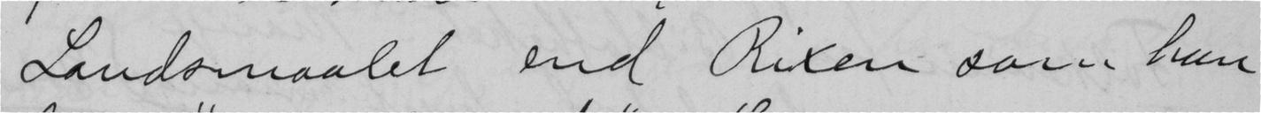Landsmaalet end Rixen som han
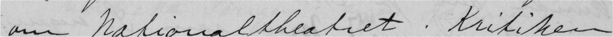om Nationaltheatret. Kritiken
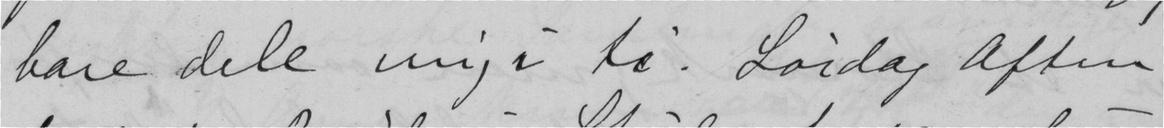bare dele mig i ti. Lørdag aften
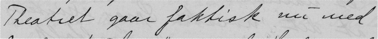Theatret gaar faktisk nu med
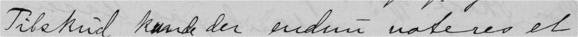Tilskud kunde der endnu noteres et
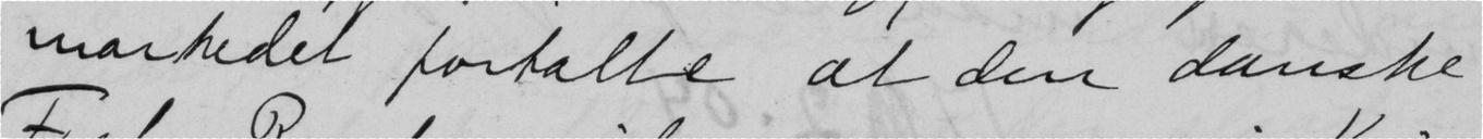markedet fortalte at den danske
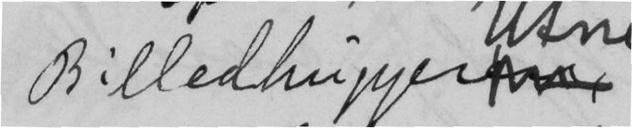Billedhugjer
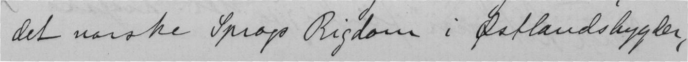det norske Sprogs Rigdom i Østlandsbygder,
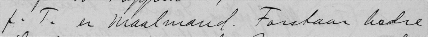f. T. er Maalmand. Forstaar bedre
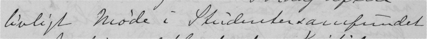livligt Møde i Studentersamfundet
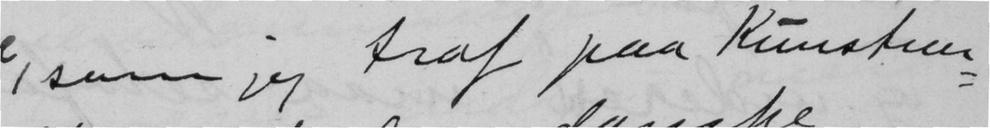som jeg traf paa Kunstner,
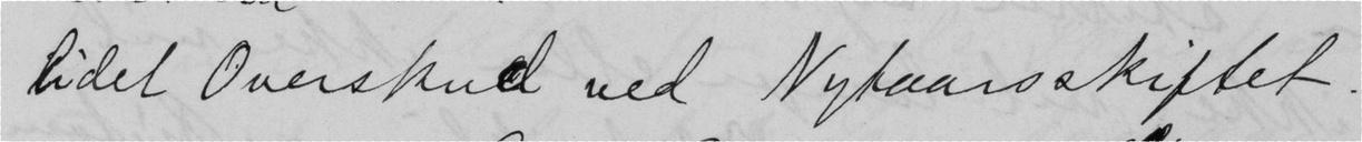lidet Overskud ved Nytaarsskiftet.
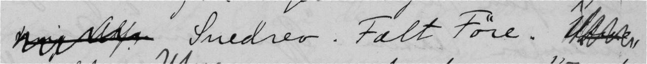Snedrev. Fælt Føre.
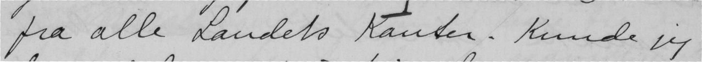fra alle Landets Kanter. Kunde jeg
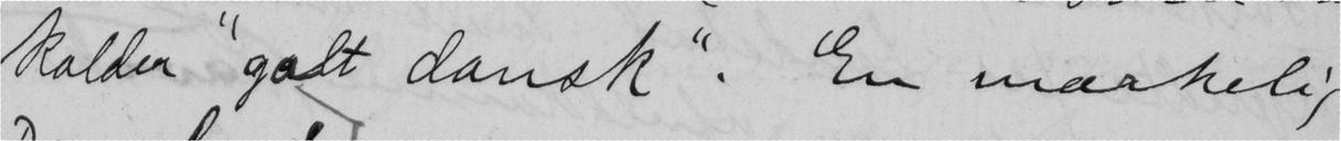kalder "godt dansk". En mærkelig
